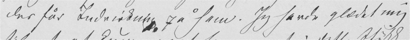der får Indvirkning på ham. Jeg havde glædet mig
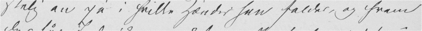stelig an på i hvilke Hænder han falder, og hvem
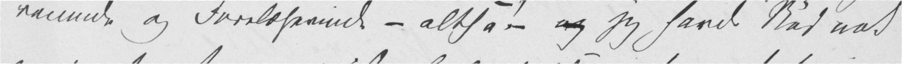veninde og Forelæserinde – altså! – og jeg havde Nød nok
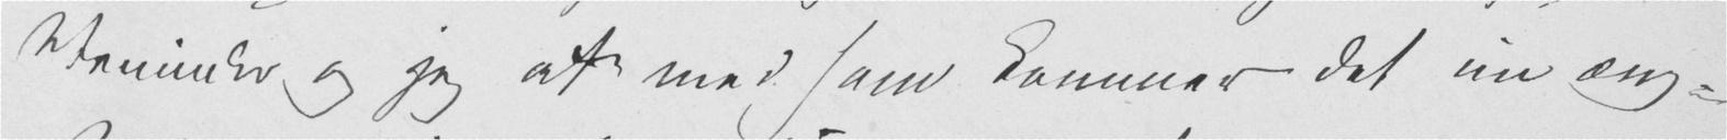Veninder og jeg at med ham kommer det nu mng-
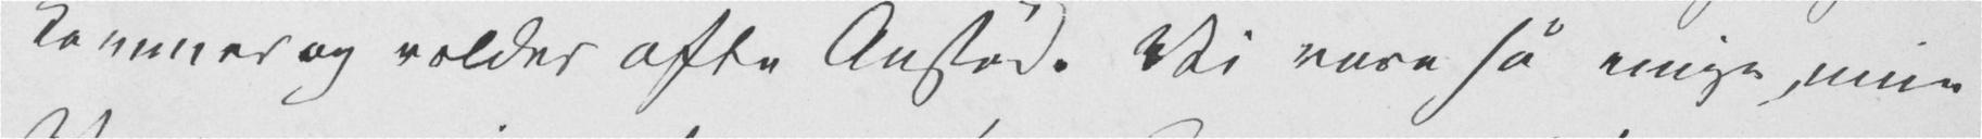kommer og volder ofte Anstød. Vi vare så enige, mine
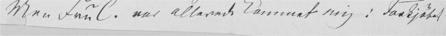Men Fru C. var allerede kommet mig i Forkjøbet
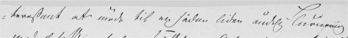teressant at møde til en sådan liden åndelig Turnering
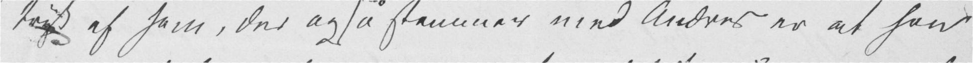tryk af ham, der også stemmer med Andres er at han
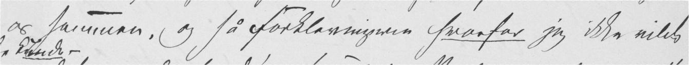os sammen, og så Forklaringerne hvorfor jeg ikke vilde
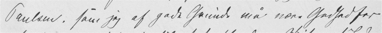Paulsen. som jeg af gode Grunde må nære Godhed for
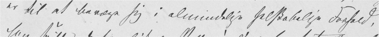er til at bevæge sig i almindelige selskabelige Forhold,
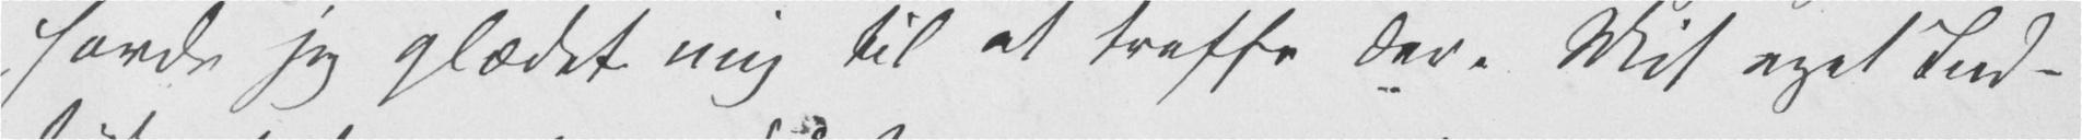havde jeg glædet mig til at treffe der. Mit eget Ind-
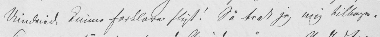Uindviede kunne forklare sligt! Så trak jeg mig tilbage.
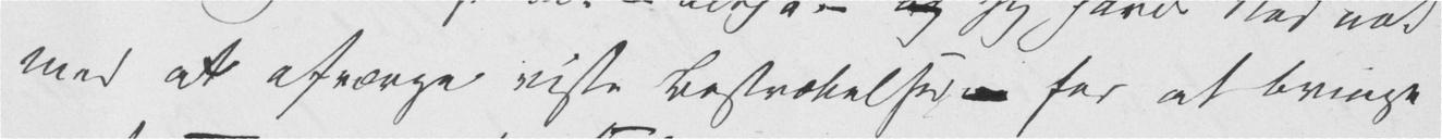med at afværge visse Bestræbelser for at bringe
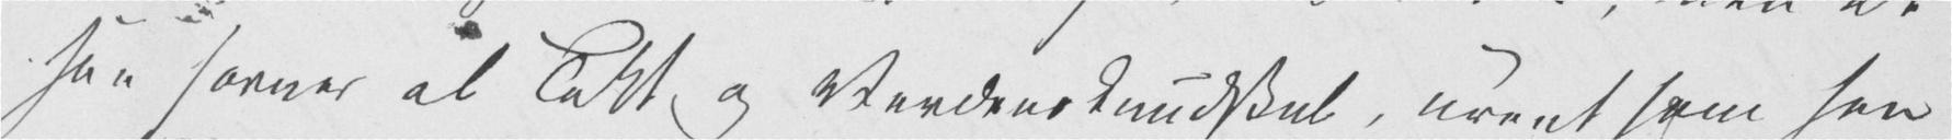han savner al Takt og Verdenskundskab, uvant som han
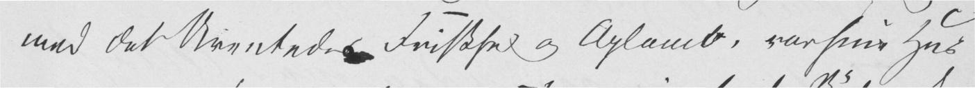med det Uventedes Friskhed og Aplomb, vor fine Hus
